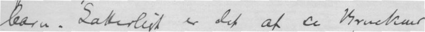barn. Latterligt er det at se Bruckner
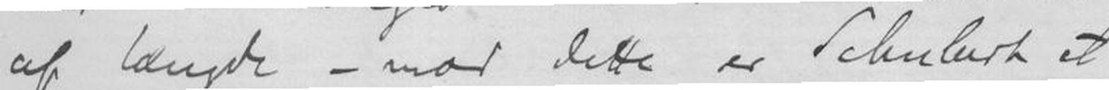af længde - mod dette er Schubert et
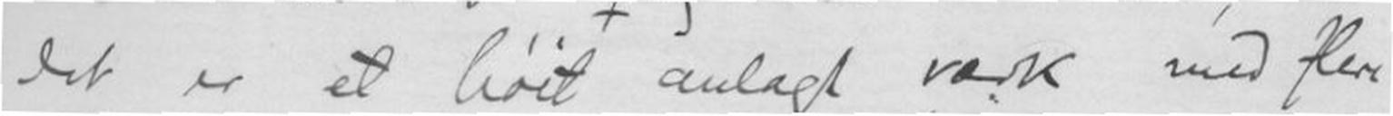det er et høit anlagt værk med flere
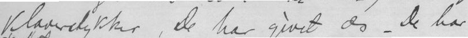klaverstykker, De har givet os. De har
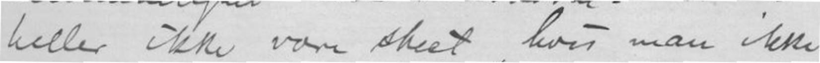heller ikke vore skeet, hvis man ikke
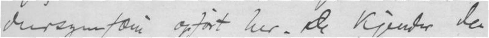dursymfoni opført her. De kjender den
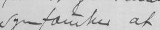en symfoniker at
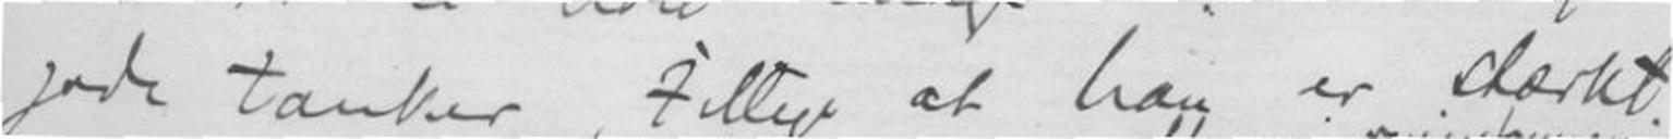gode tanker, tillige at han er stærkt
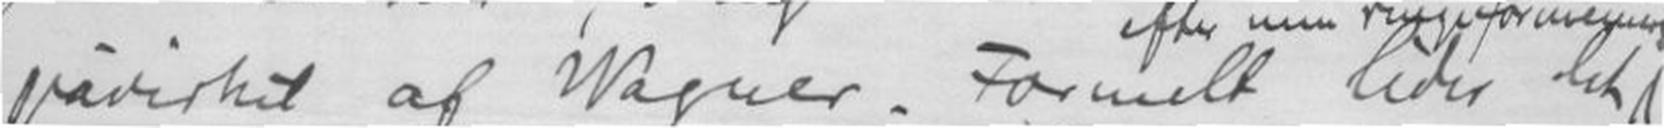påvirket af Wagner. Formelt lider det
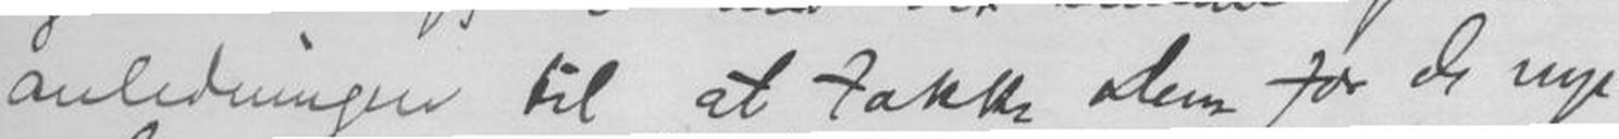anledningen til at takke Dem for de nye
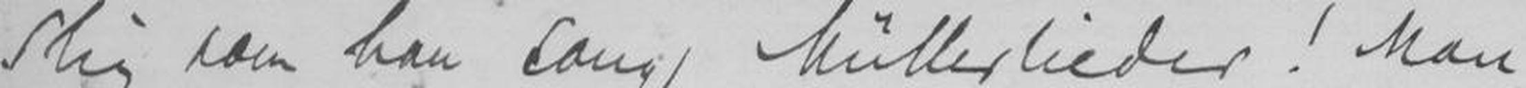Slig som han sang Müllerlieder! Man
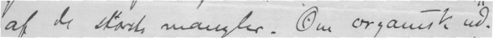af de største mangler. Om organisk ud-
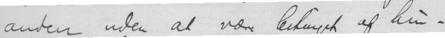anden uden at være betinget af hin-
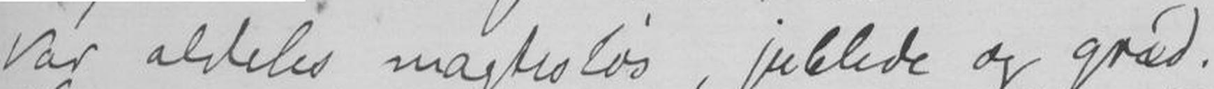var aldeles magtesløs, jublede og græd.
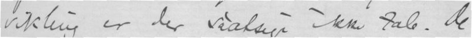vikling er der såatsige ikke tale. De
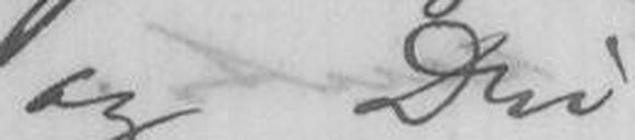og Din
